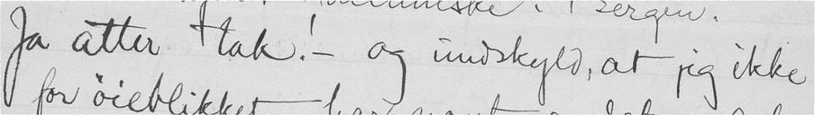Ja atter tak! - og undskyld, at jeg ikke
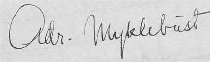Adr. Myklebust
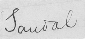Sandal
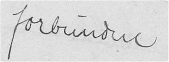forbundne
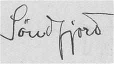Søndfjord
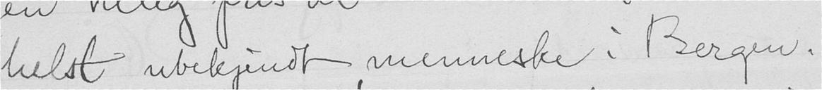helst ubekjendt menneske i Bergen.
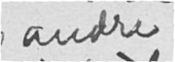andre
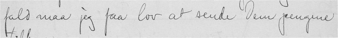fald maa jeg faa lov at sende Dem pengene
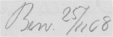Besv. 25/11 08
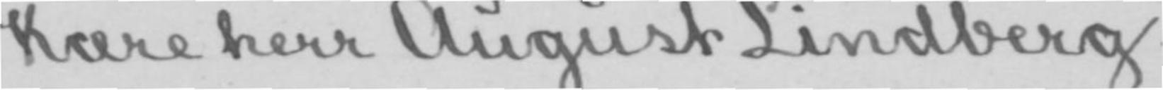Kære herr August Lindberg.
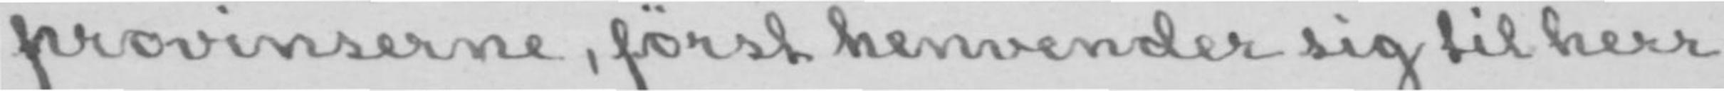provinserne, først henvender sig til herr
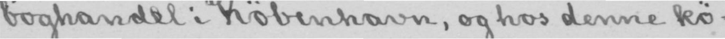boghandlel i København, og hos denne kø-
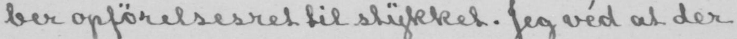ber opførelsesret til stykket. Jeg véd at der
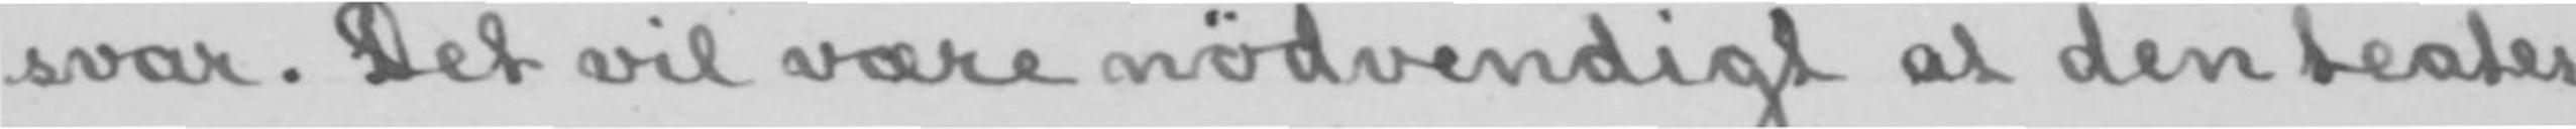svar. Det vil være nødvendigt at den teater
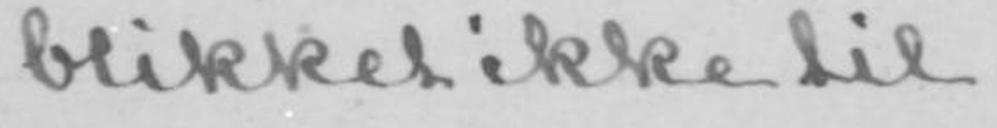blikket ikke til.
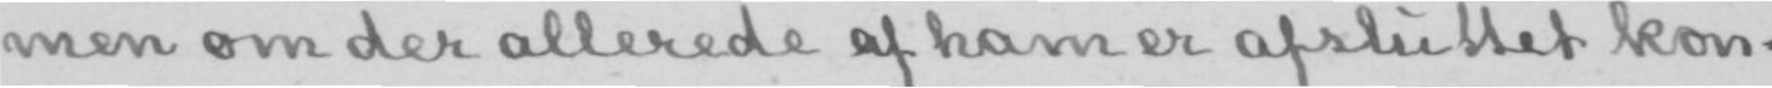men om der allerede af ham er afsluttet kon-
